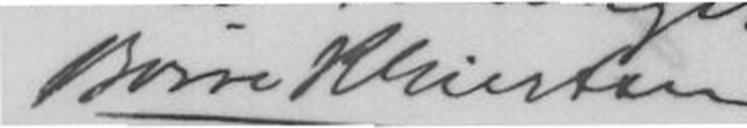Børre R Giertsen
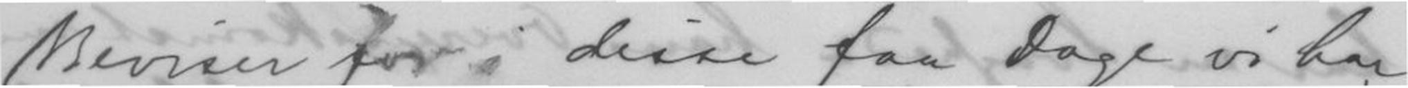Beviser for i disse faa Dage vi har
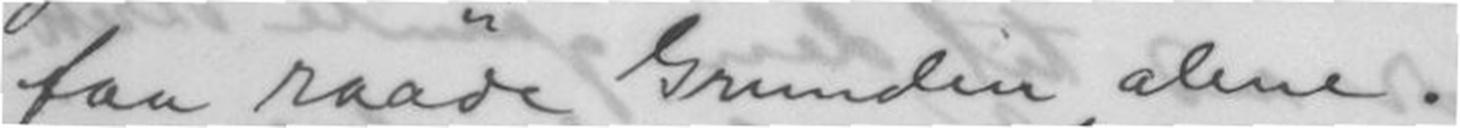faa raade Grunden alene.
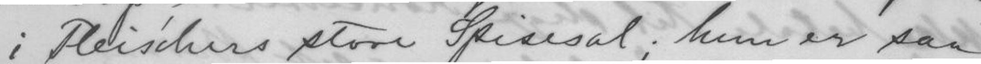i Fleis'chers store Spisesal; hun er saa
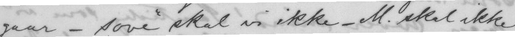gaar - sove skal vi ikke _ M. skal ikke
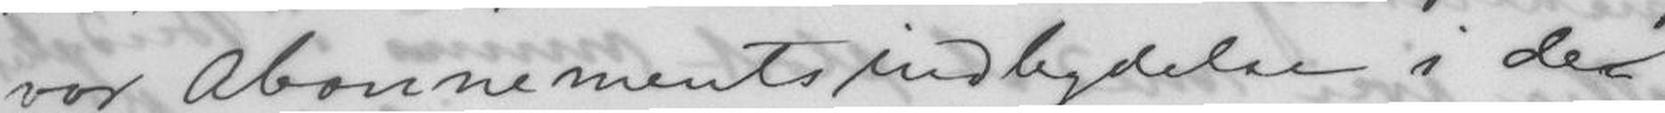vor Abonnementsindbydelse i de
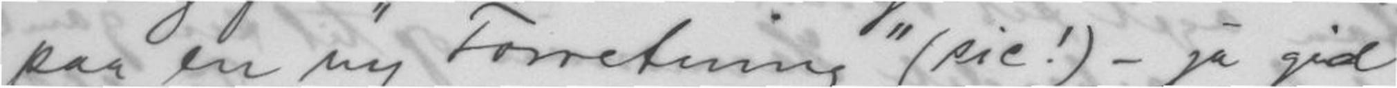paa en ny Forretning (sic!) - ja gid
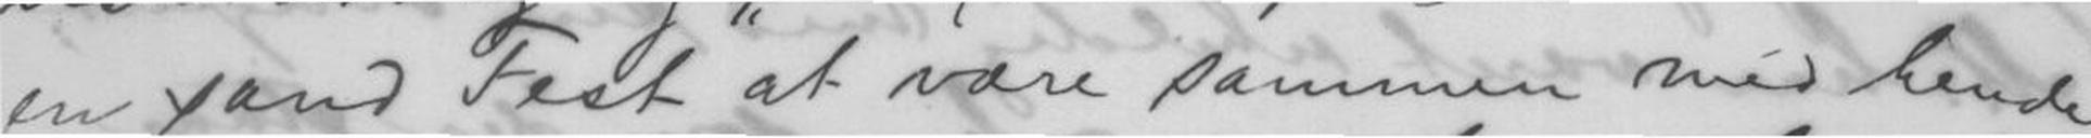en sand Fest at være sammen med hende
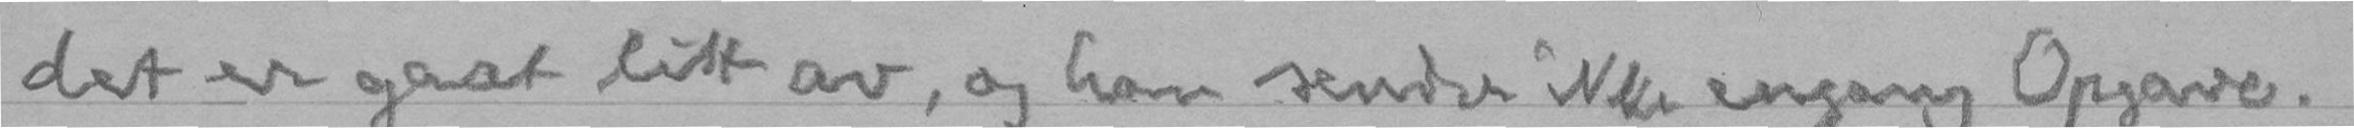det er gaat litt av, og han sender ikke engang Opgave.
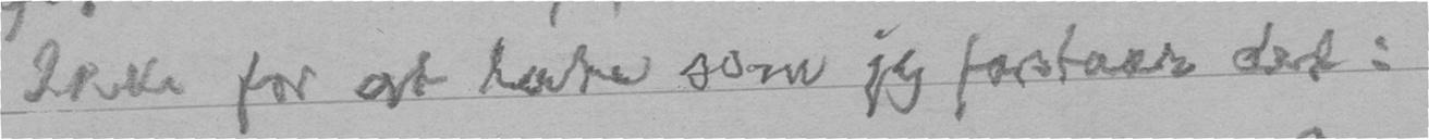Ikke for at lade som jeg forstaar det:
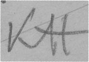KH
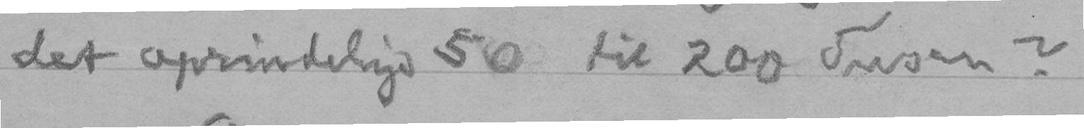det oprindelige 50 til 200 Tusen?
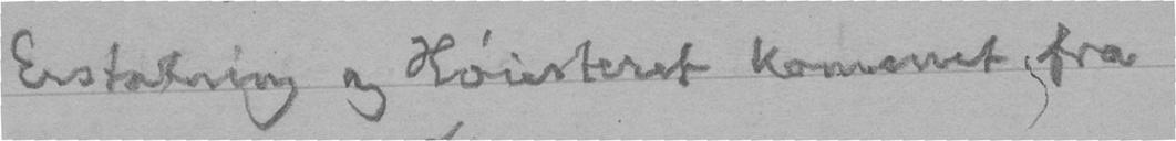Erstatning og Høiesteret kommet fra
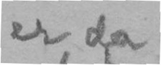er da
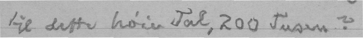til dette høie Tal, 200 Tusen?
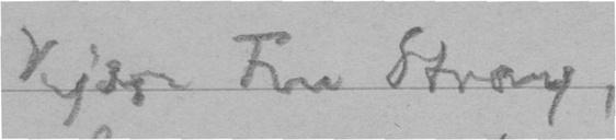Kjære Fru Stray,
